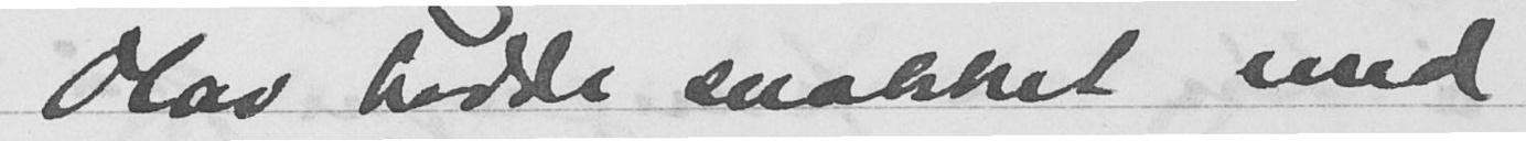Olav hadde snakket med
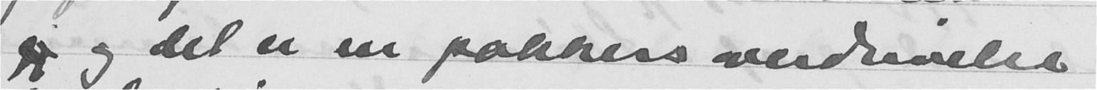og det er en pokkers overdrivelse
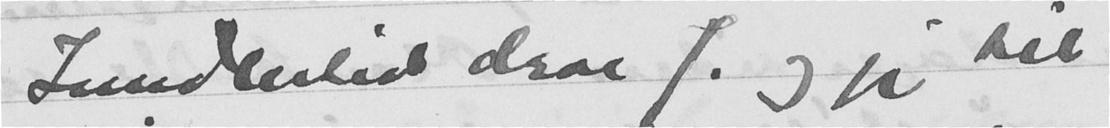Imidlertid drar J. og jeg til
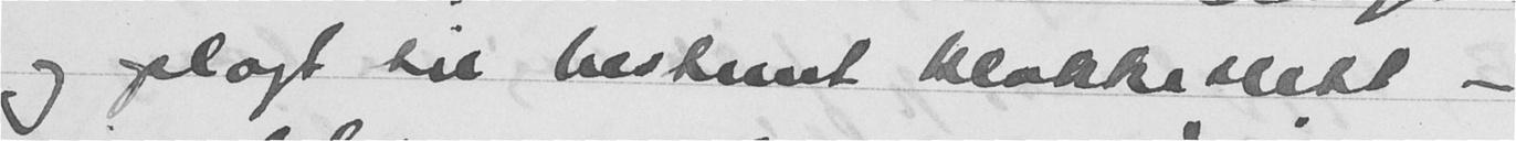og oplagt til bestemt klokkeslett -
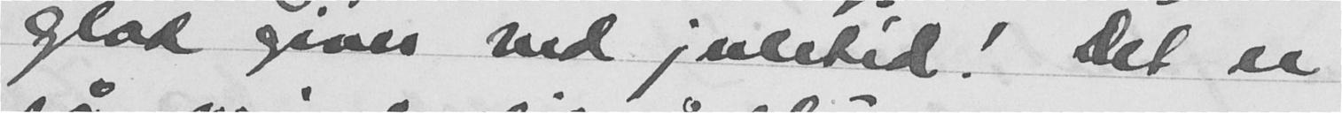glad giver ved juletid! Det er
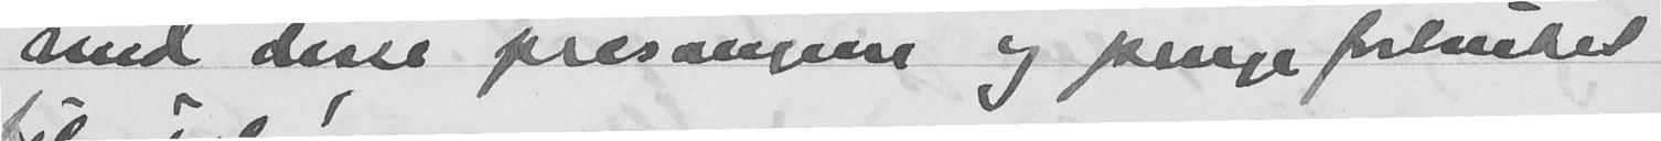med disse presangene og pengeforbruket
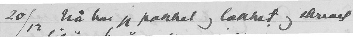20/12 Nå har jeg pakket og takket og skrevet
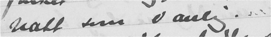natt som vanlig.
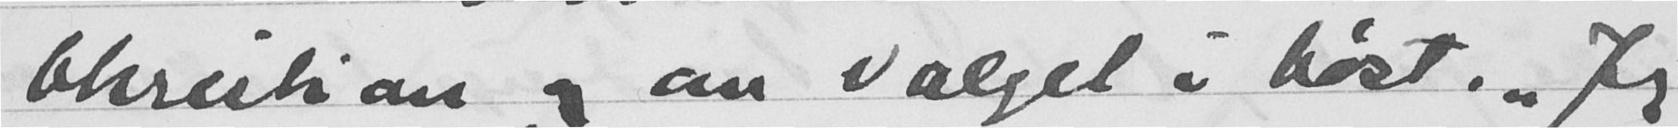Christian og om valget i høst. "Jeg
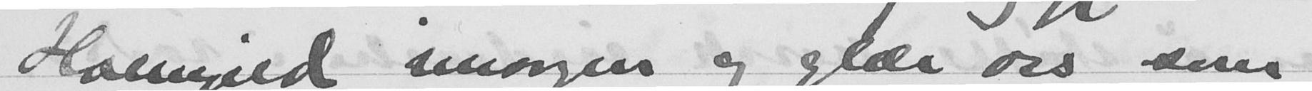Holmgild imorgen og glær oss som
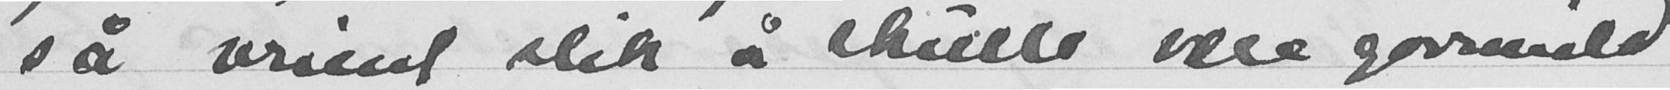så vrient slik å skulle være gavmild
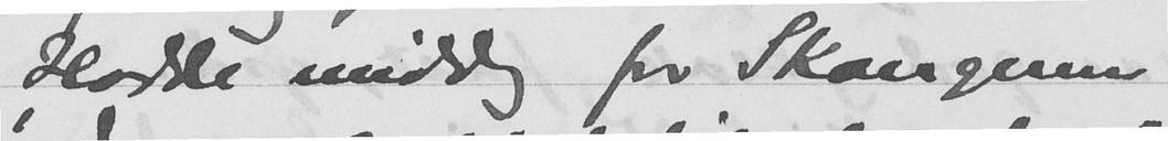Hadde middag for Skaugum
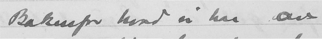Bakenfor hvad vi har av
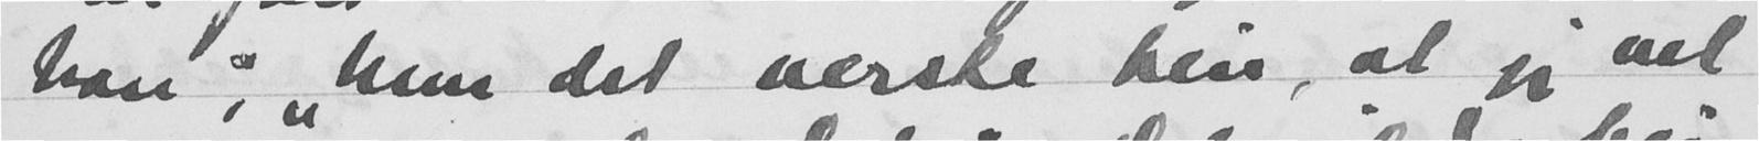han; "men det verste blir, at jeg vel
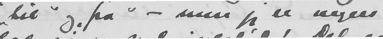"til" og "fra" - men jeg er ingen
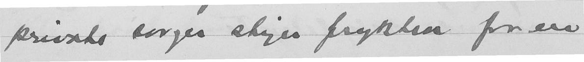private sorger stiger frykten for en
